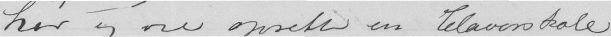her og vil oprette en Claverskole
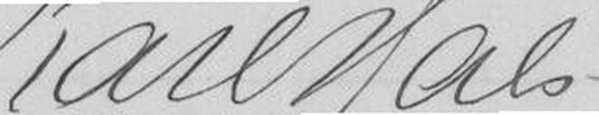Karl Hals
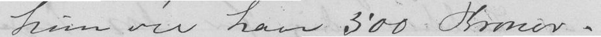hun vil have 500 Kroner
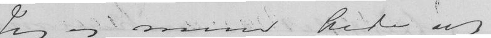Jeg og mine bede at
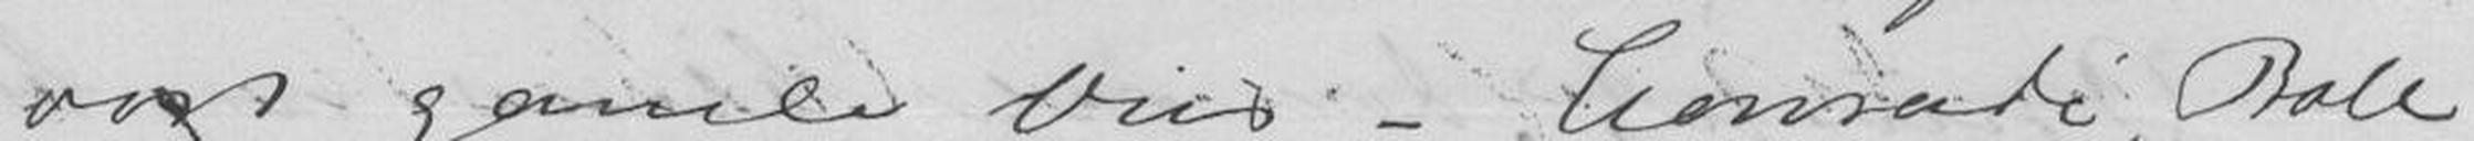vort gamle viis - Conradi, Bole
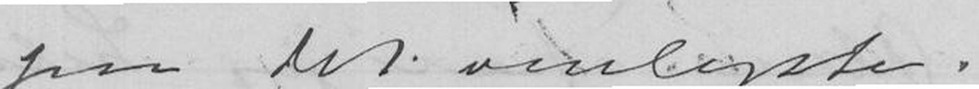paa det venligste
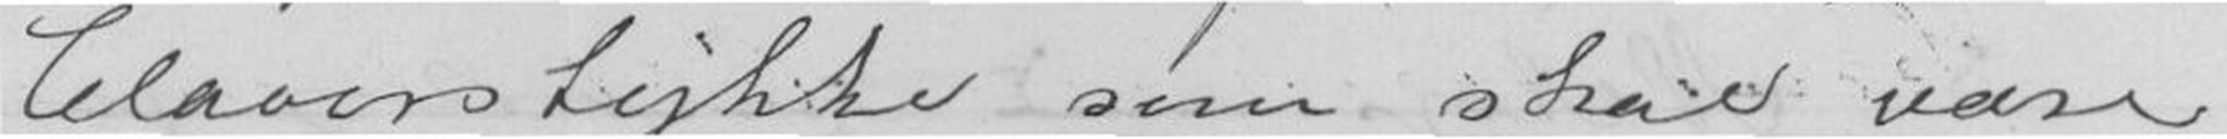Claverstykke som skal være
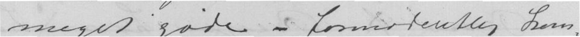meget gode - formodentlig kom-
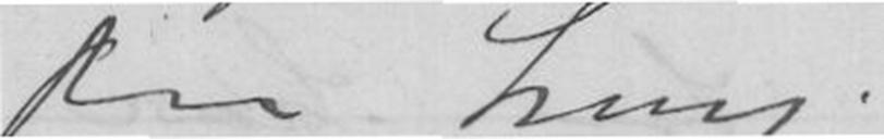Din heng.
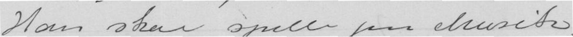Han skal spille paa Musik-
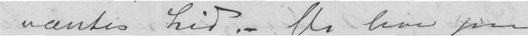væntes hid. - Vi leve paa
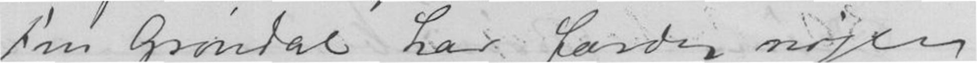Fru Grøndal har færdig nogle
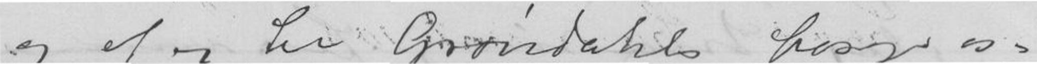og af og til Grøndahls besøge os.
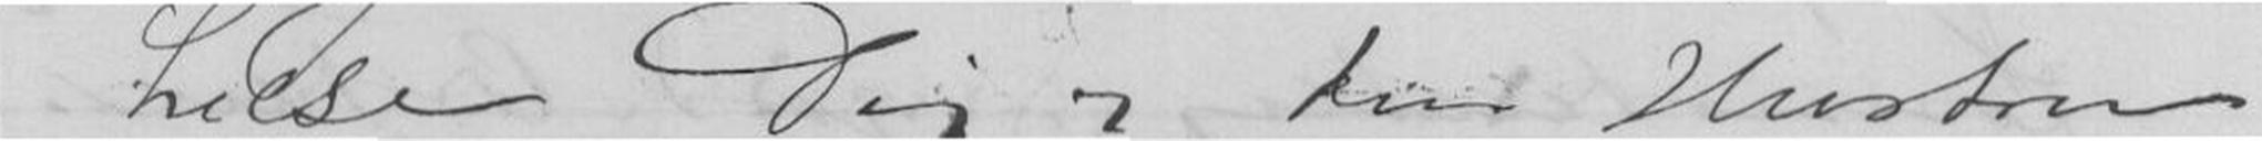hilse Dig og Din Hustru
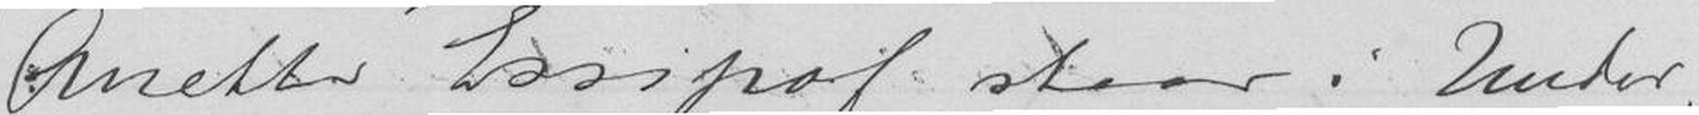Anette Essipof staar i Under-
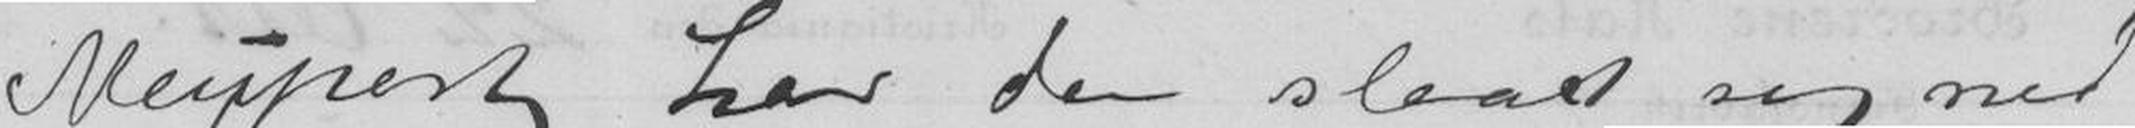Neupert har da slaaet sig ned
